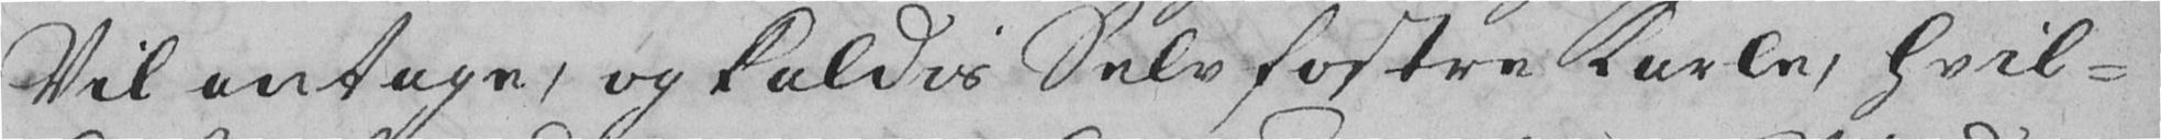Vil antage, og kaldis selv fostre Karle, hvil-
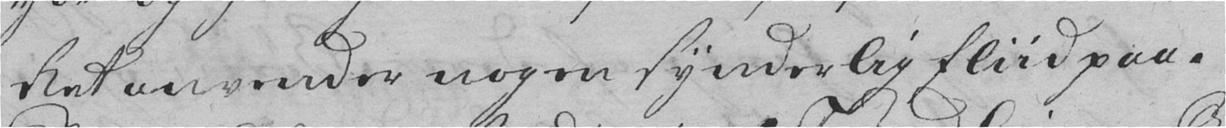Ret anvender nogen synderlig fliid paa.
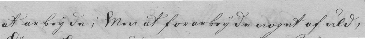at arbeyde; Men at forarbeyde noget af uld,
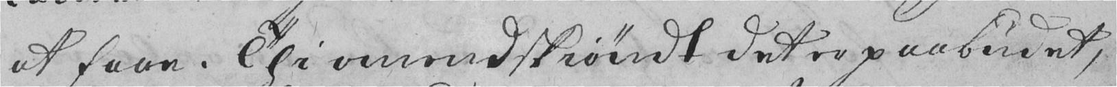at faae. Thi omendskiøndt det er paabudet,
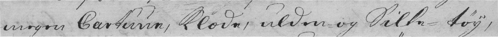megen Cartuun, Klæde, ulden- og Silke-tøy,
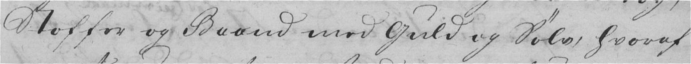Stoffer og Baand med Guld og Sølv, hvoraf
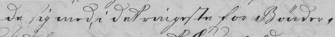de sig med, i det ringeste for Bønder.
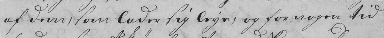af dem, som lader sig leye, og for nogen tid
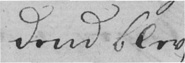dend blev
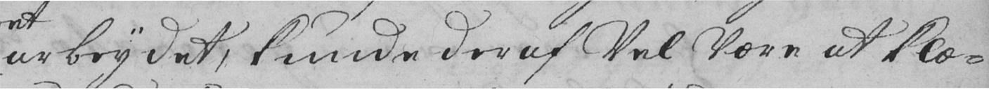arbeydet, kunde deraf Vel Være at klæ-
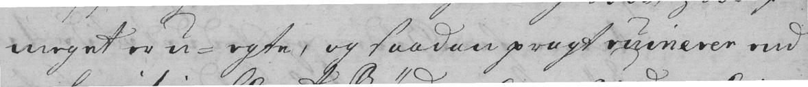meget er uegte, og saadan pragt ruinerer end
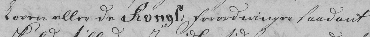Loven eller de Kongl. Forordninger saadant
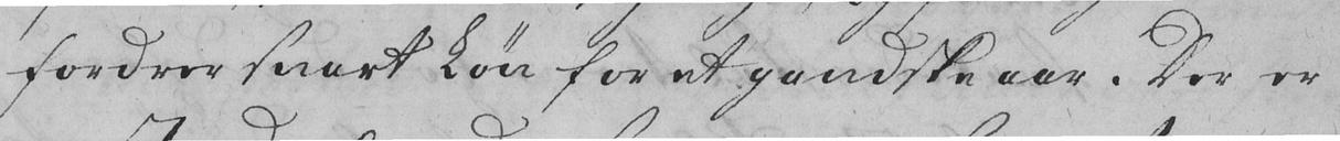fordrer snart Løn for et gandske aar. Der er
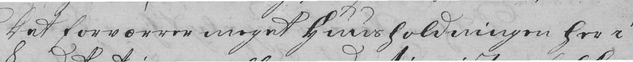Det forværrer meget Huusholdningen her i
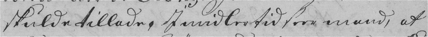skulde tillade. Imidlertid seer mand, at
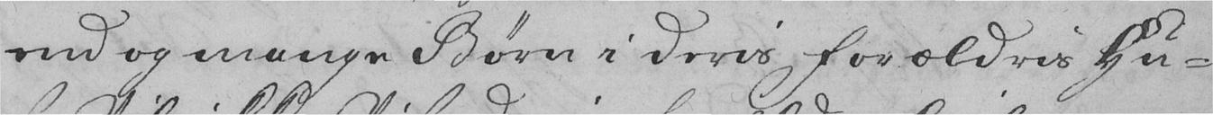end og mange Børn i deris Forældres Hu-
